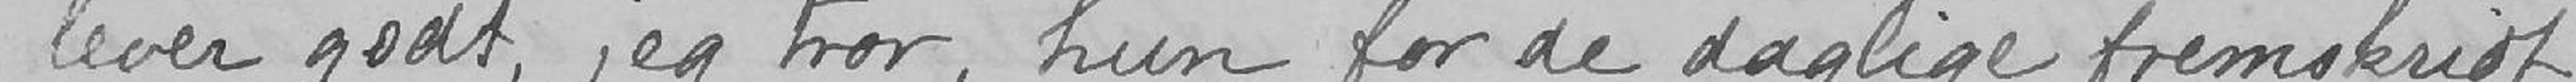lever godt, jeg tror, hun for de daglige fremskridt
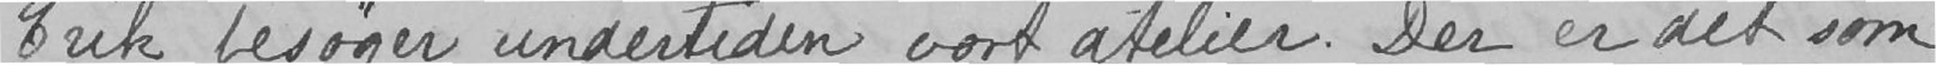Erik besøger undertiden vort atelier. Der er det som
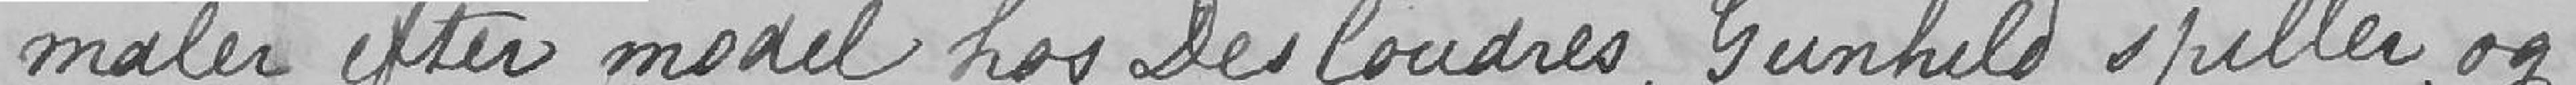maler efter model hos Desloudres, Gunhild spiller, og
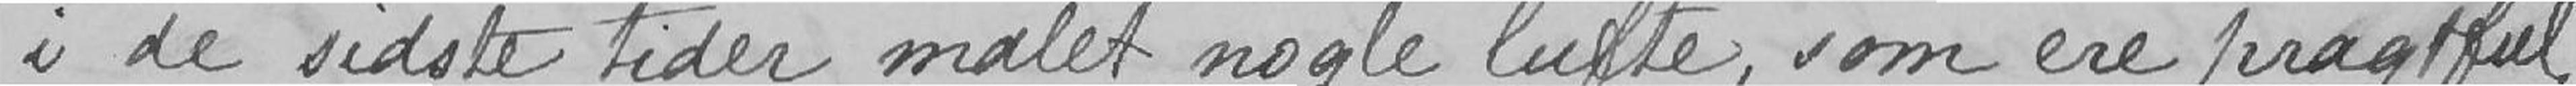i de sidste tider malet nogle lufte, som ere pragtfulde.
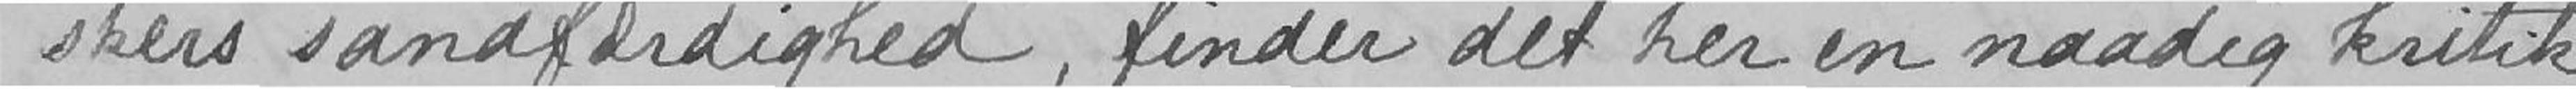skers sandfærdighed, finder det her en naadig kritik.
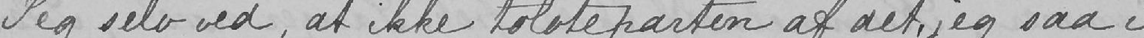Jeg selv ved, at ikke tolvteparten af det, jeg saa i
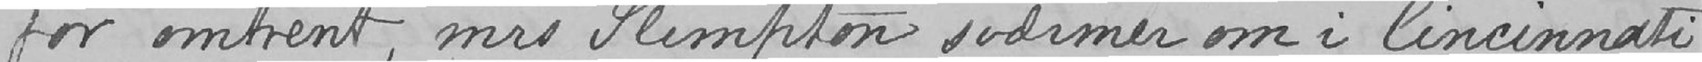før omtrent, mrs Plimpton sværmer om i Cincinnati
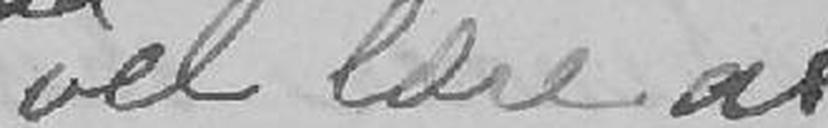vel lære at
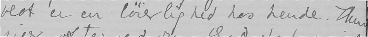blot er en løierlighed hos hende. Hun
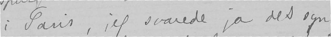i Paris, jeg svarede ja det syn-
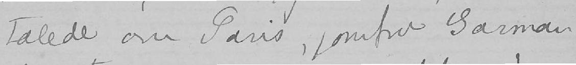talede om Paris, jomfru Garman
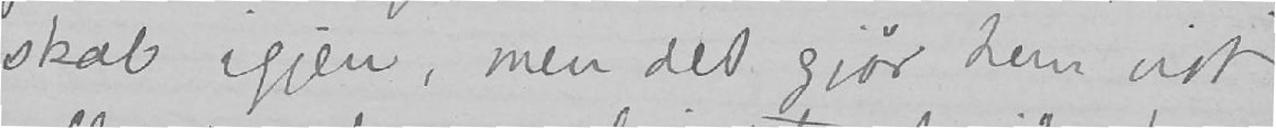skab igjen, men det gjør hun vist
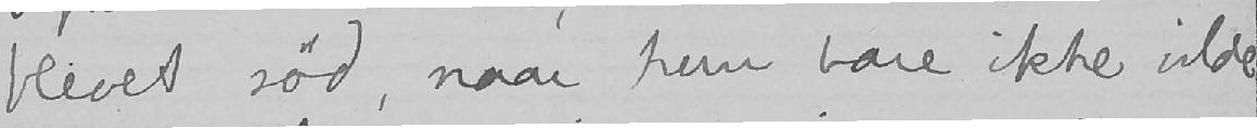blevet sød, naar hun bare ikke vilde
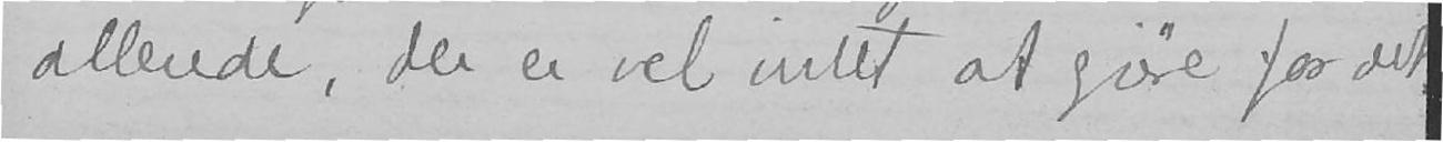allerede, der er vel intet at gjøre for det.
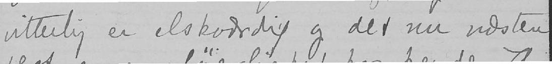vitterlig er elskværdig og det nu næsten
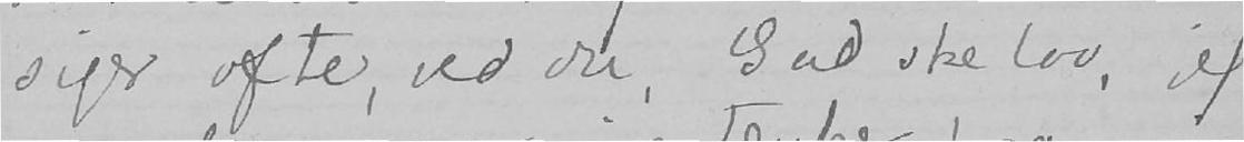siger ofte, ved du, Gud ske lov, jeg
